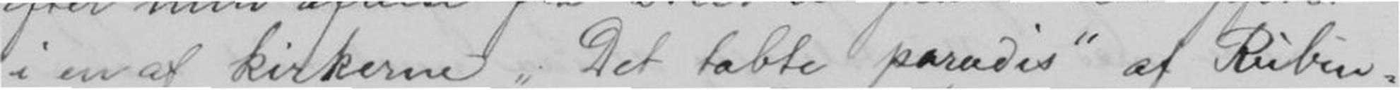i en af kirkene "Det tabte paradis" af Rubin-
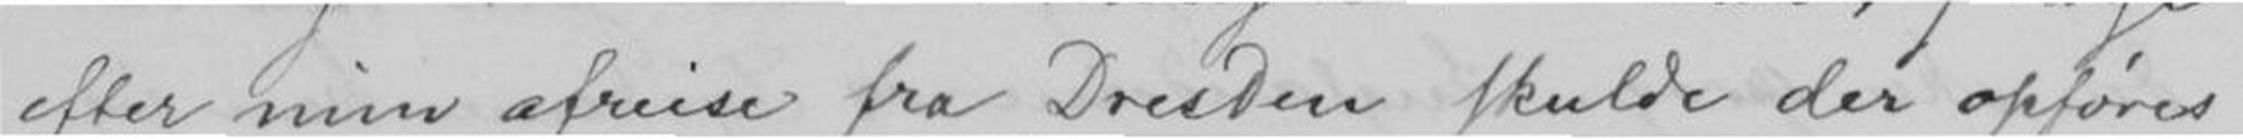efter min afreise fra Dresden skulde der opføres
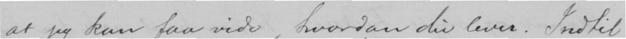at jeg kan faa vide, hvordan du lever. Indtil
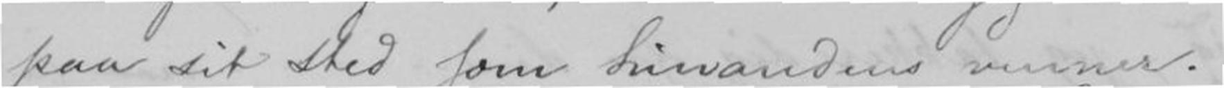paa sit sted som hinandens venner.
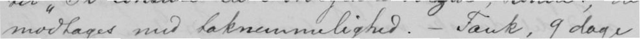modtages med taknemlighed. - Tænk, 9 dage
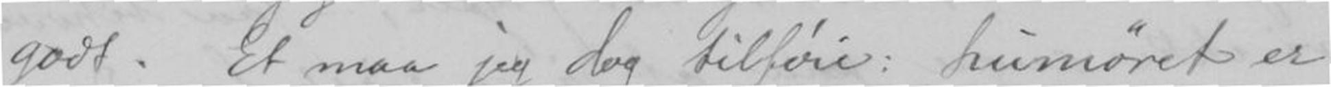godt. Et maa jeg dog tilføre: humøret er
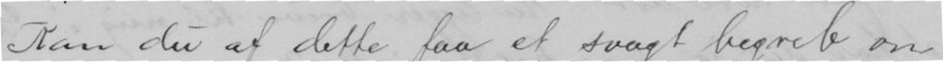Kan du af dette faa et svagt begreb om
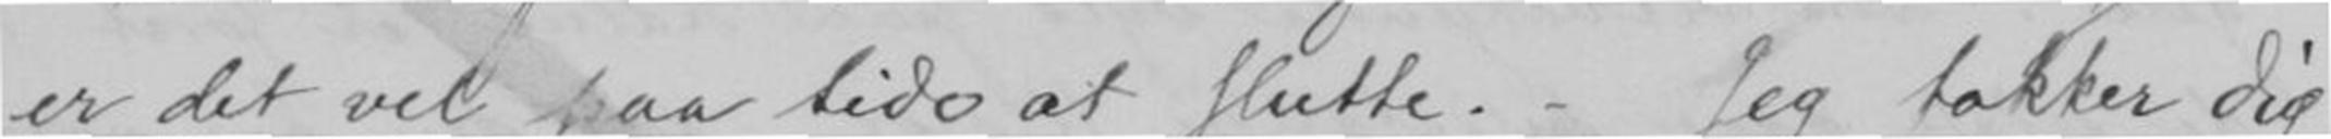er det vel paa tide at slutte. - Jeg takker dig
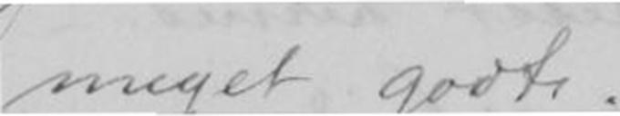meget godt. -
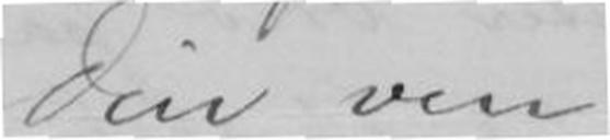Din ven
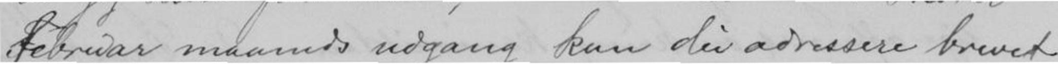februar maaneds udgang kan du adressere brevet
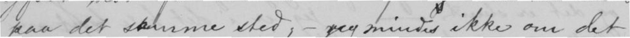paa det samme sted, - jeg mindes ikke om det
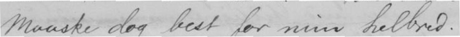Maaske dog best for min helbred.
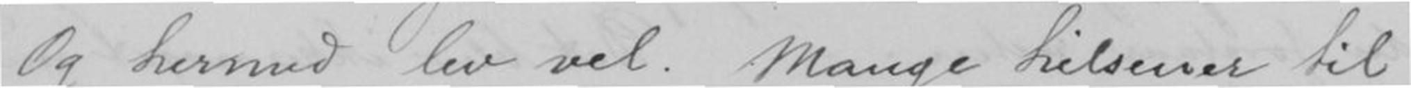Og hermed lev vel. Mange hilsener til
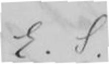P. s.
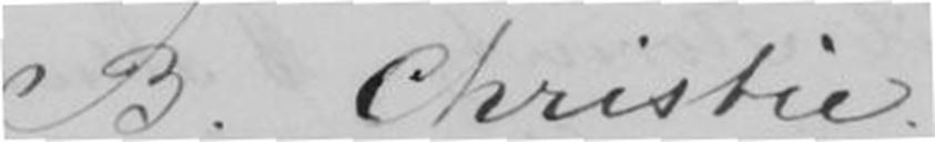B Christie
